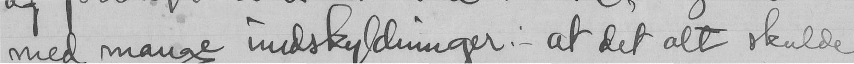med mange undskyldninger: - at det alt skulde
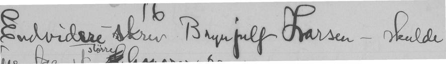Endvidere skrev Brynjulf Larsen - skulde
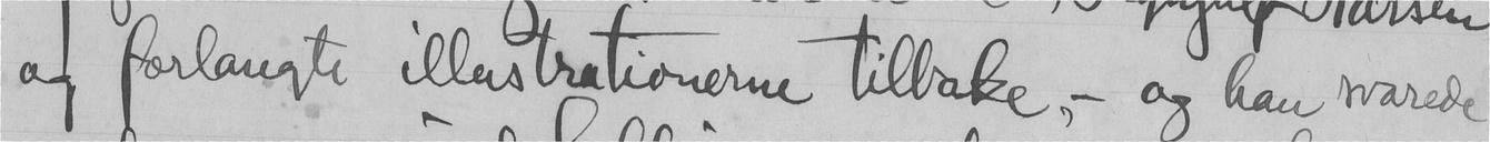og forlangte illerstrationerne tilbake, - og han svarede
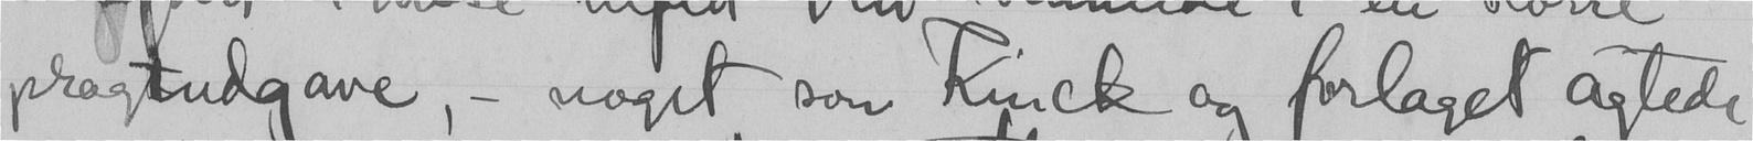pragtudgave, - noget som Kinck og forlaget agtede
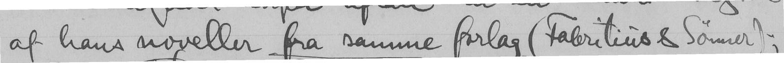af hans noveller fra samme forlag (Fabritiuss Sømer);
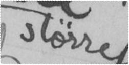større
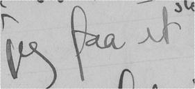jeg faa et
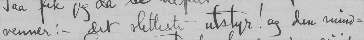venner, - det sletteste utstyr! og den mind-
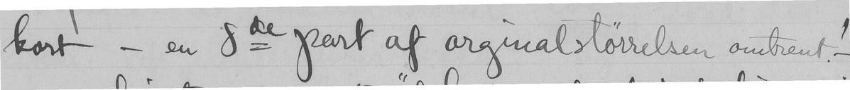kort - en 8de part af orginalstørrelsen omtrent! -
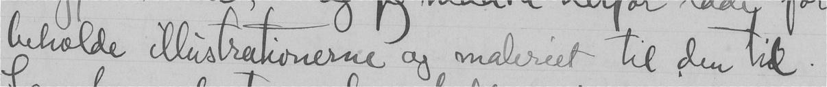beholde illustrationerne og maleriet til den tid.
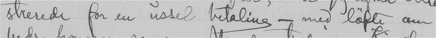strerede for en ussel betaling - med løfte om
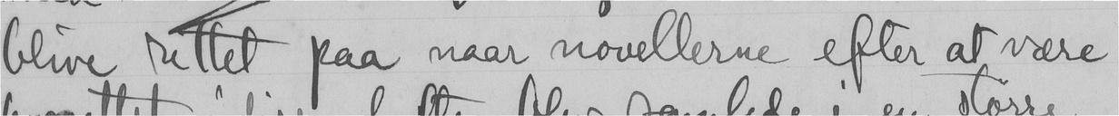blive rettet paa naar novellerne efter at være
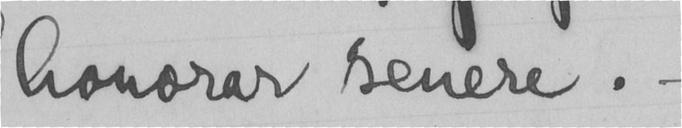honorar senere. -
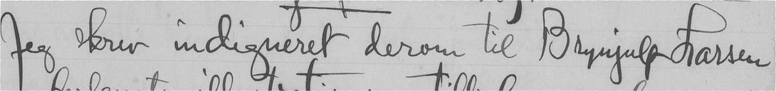Jeg skrev indigneret derom til Brynjulf Larsen
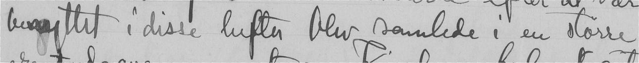benyttet i disse hefter blev samlede i en større
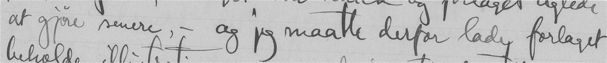at gjøre senere, - og jeg maate derfor lade forlaget
